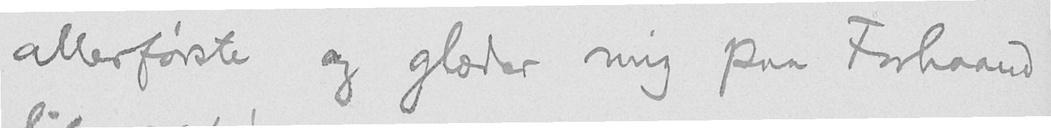allerførste og glæder mig paa Forhaand
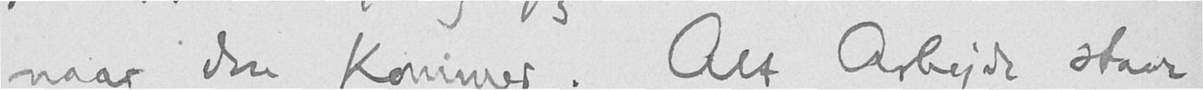naar den kommer. Alt Arbejde staar
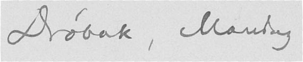Drøbak, Mandag
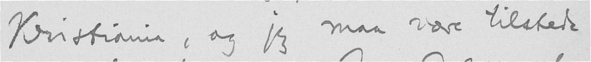Kristiania, og jeg maa være tilstede
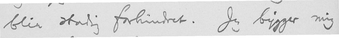blir stadig forhindret. Jeg bygger mig
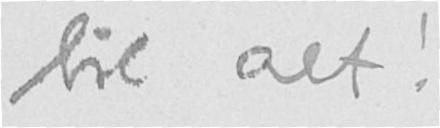til alt!
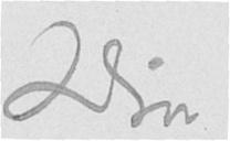Din
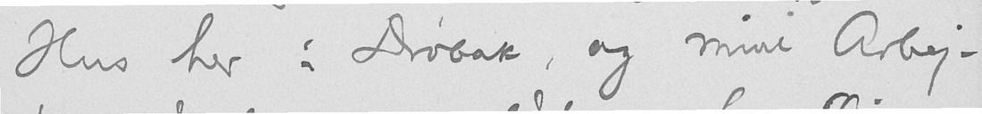Hus her i Drøbak, og mine Arbej-
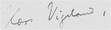Kære Vigeland,
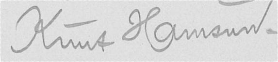Knut Hamsun.
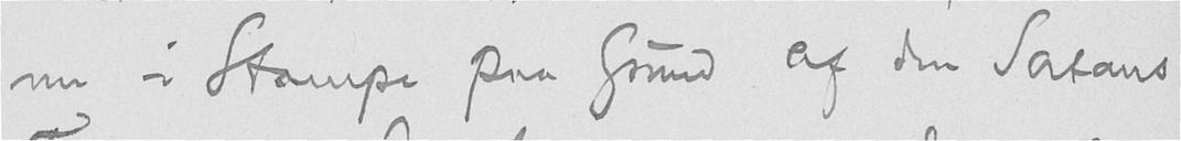nu i Stampe paa Grund af den Satans
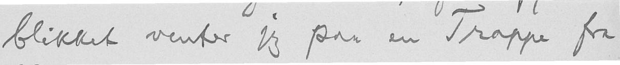blikket venter jeg paa en Trappe fra
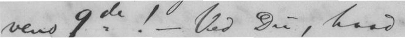vens 9 de! - Ved Du, hvad
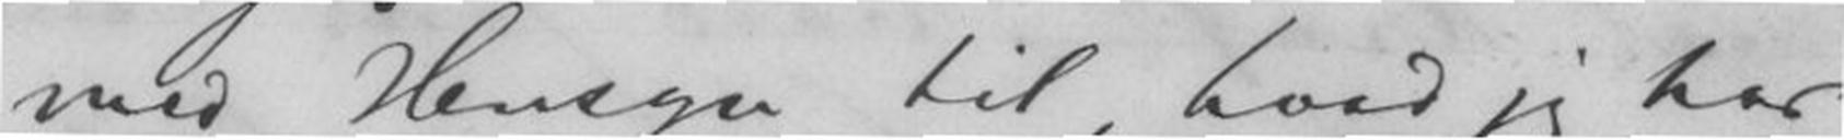med Hensyn til, hvad jeg har
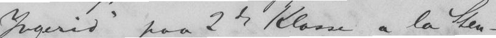"Ingerid" paa 2den Klasse a la Sten-
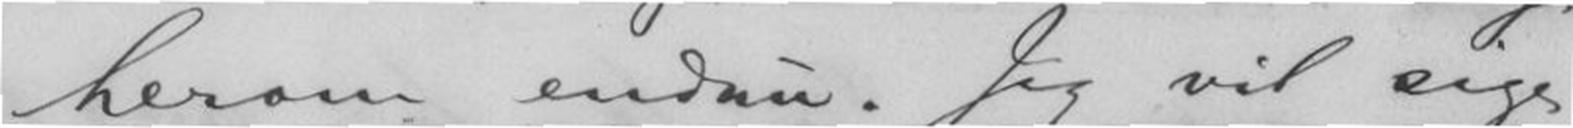herom endnu. Jeg vil sige,
